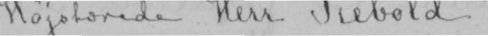Højstærede Herr Siebold!
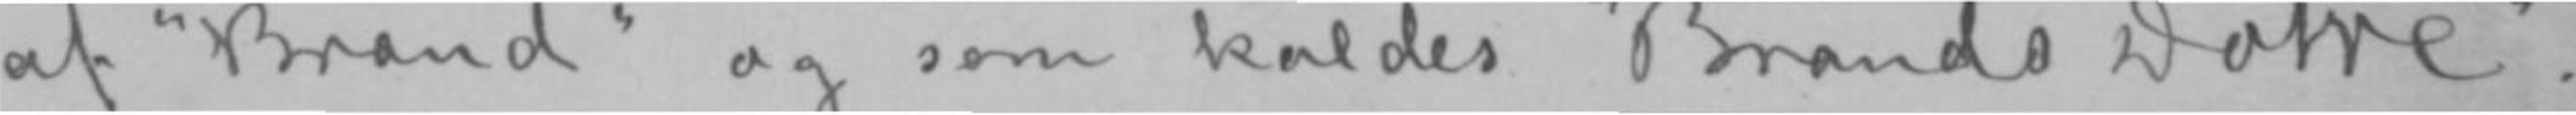af «Brand» og som kaldes «Brands Døtre».
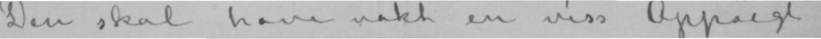Den skal have vakt en viss Oppsigt;
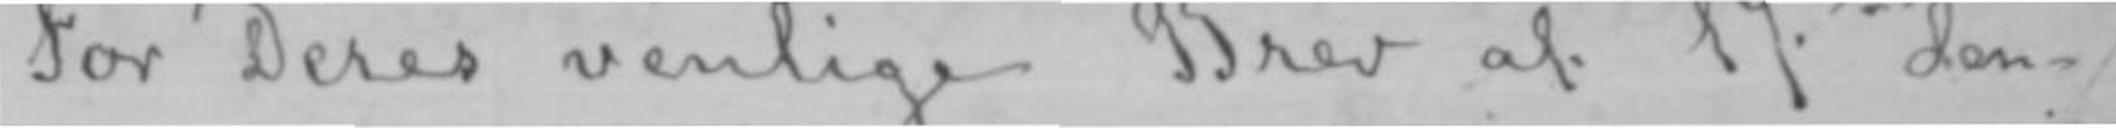For Deres venlige Brev af 17de den-
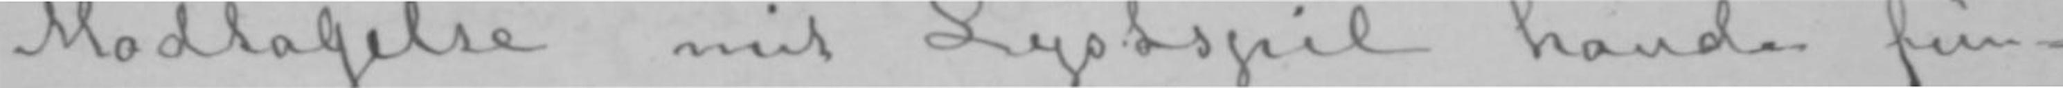Modtagelse mit Lystspil havde fun-
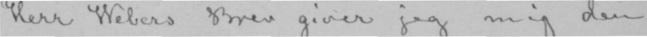Herr Webers Brev giver jeg mig den
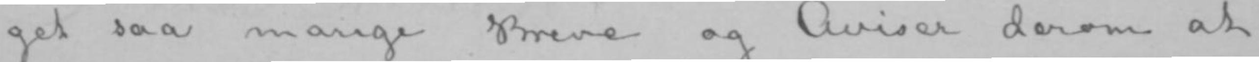get saa mange Breve og Aviser derom at
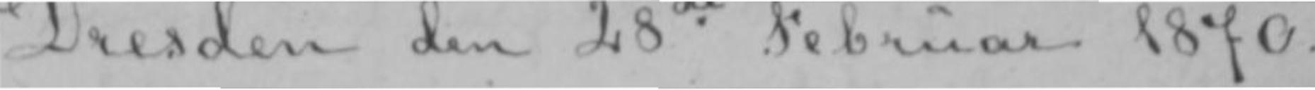Dresden den 28de Februar 1870.-
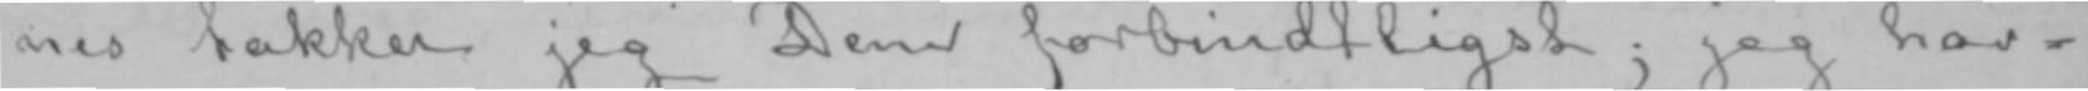nes takker jeg Dem forbindtligst; jeg hav-
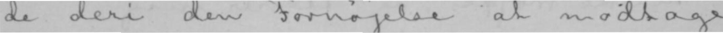de deri den Fornøjelse at modtage
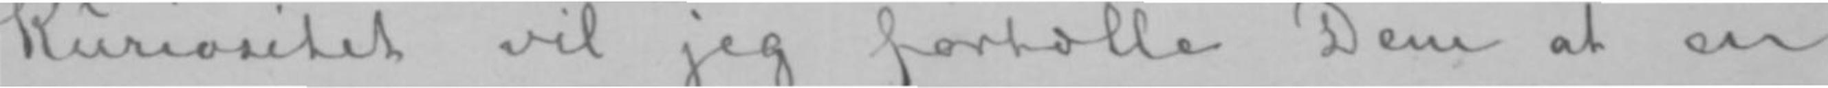Kuriositet vil jeg fortælle Dem at en
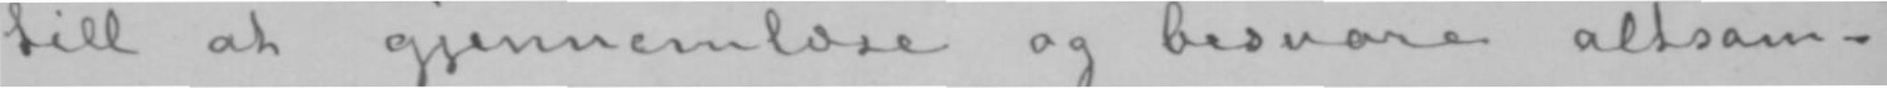till at gjennemlæse og besvare altsam-
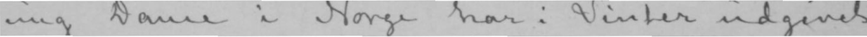ung Dame i Norge har i Vinter udgivet
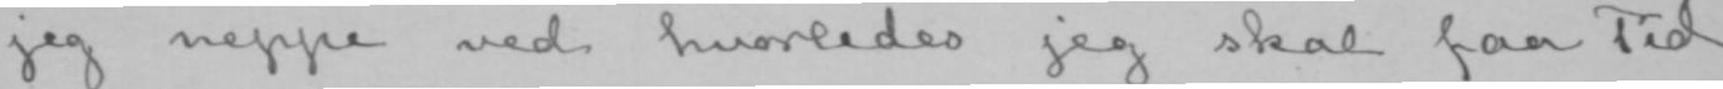jeg neppe ved hvorledes jeg skal faa Tid
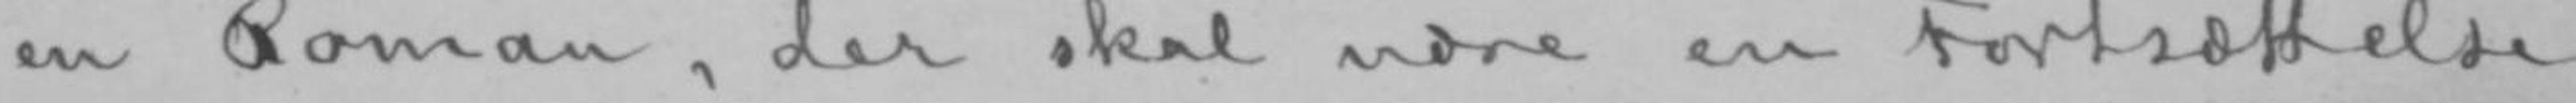en Roman, der skal være en Fortsættelse
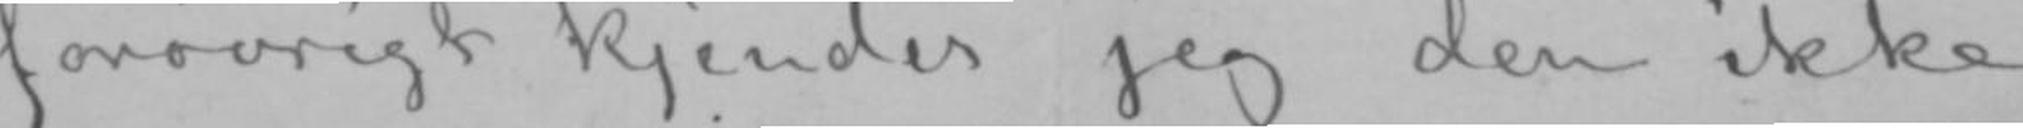forøvrigt kjender jeg den ikke.
